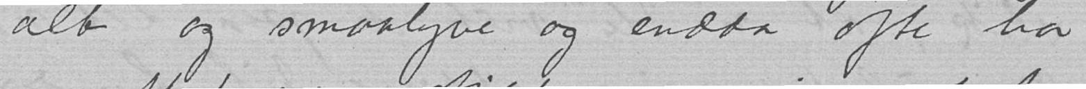alt og smaalyve og endda ofte har
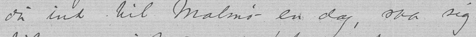du ind til Malmø en dag, saa sig
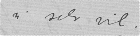vi selv vil.
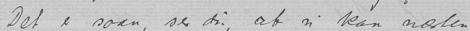Det er saan, ser du, at vi kan næsten
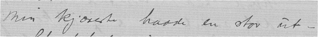Min kjæreste hadde en stor ut-
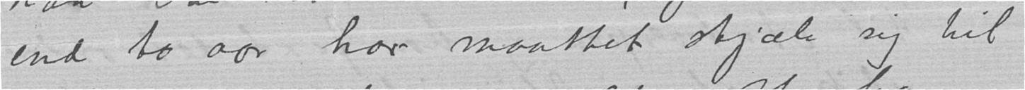end to aar har maattet stjæle sig til
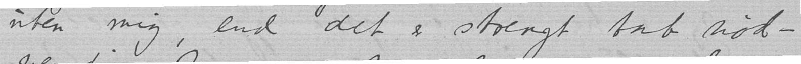uten mig, end det er strengt tat nød-
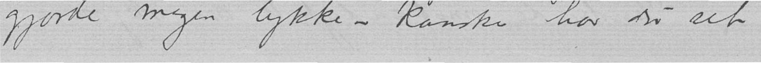gjorde megen lykke - kanske har du set
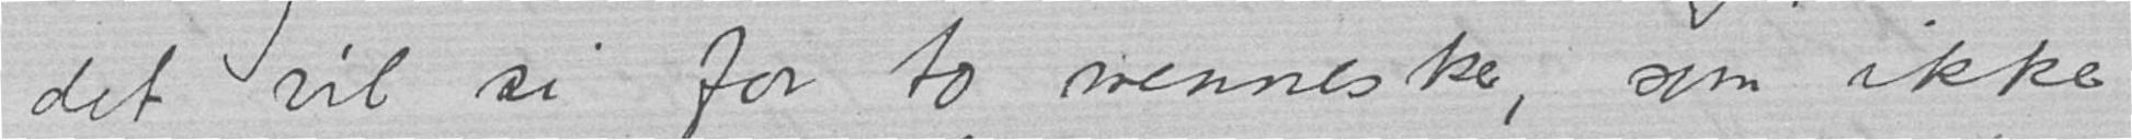det vil si for to menneske, som ikke
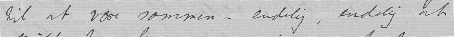til at være sammen - endelig, endelig at
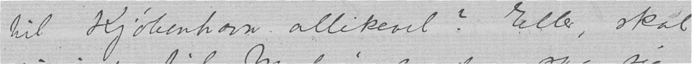til Kjøbenhavn allikevel? Eller, skal
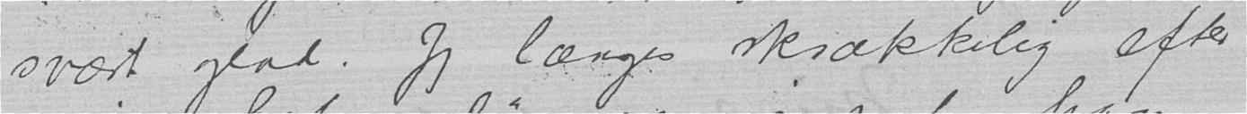svært glad. Jeg længes skrækkelig efter
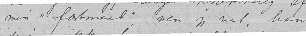min "fæstmand", men jeg vet, han
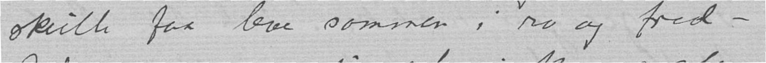skulle faa leve sammen i ro og fred -.
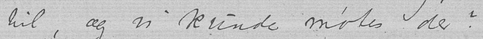til, og vi kunde møtes der?
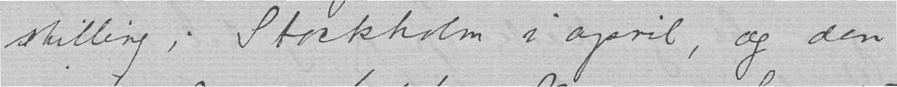stilling i Stockholm i april, og den
